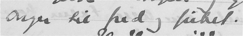onger til fred og frihet.
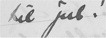til jul!
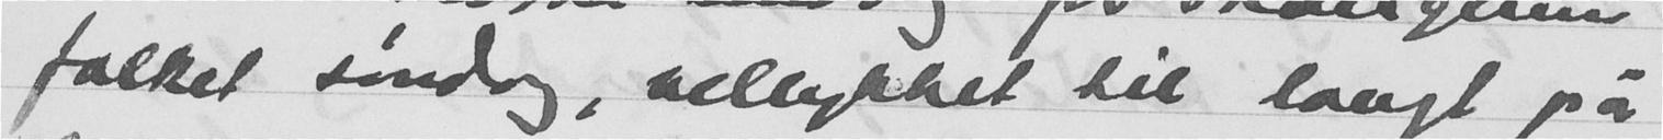folket søndag, vellykket til langt på
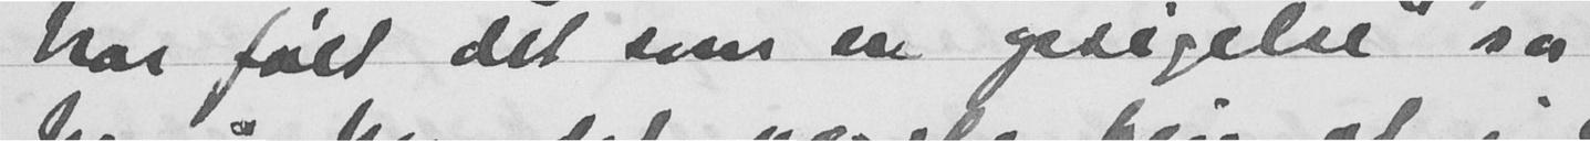har fått det som en opsigelse" sa
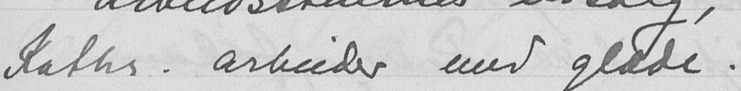Kathr. arbeider med glæde.
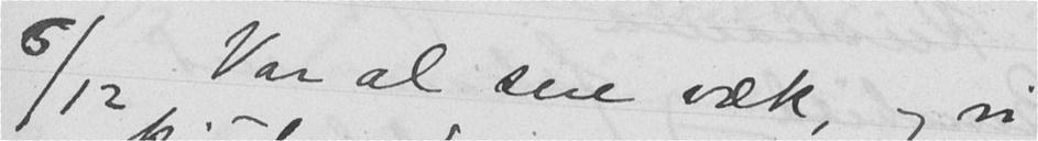6/12 Var al sne væk, og vi
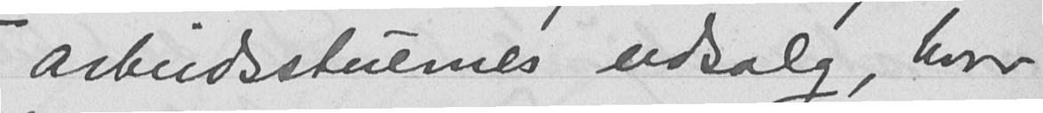arbeidsstuernes udsalg, hvor
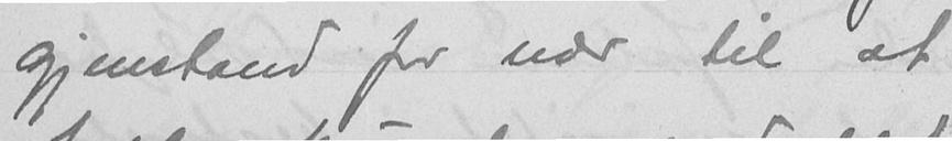gjenstand for nær til at
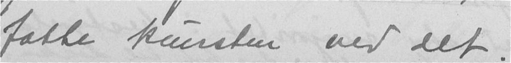fatte kunsten ved det.
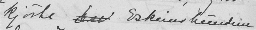kjørte vae Eskimohundene
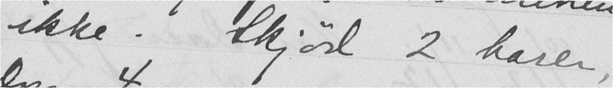ikke. Skjød 2 harer,
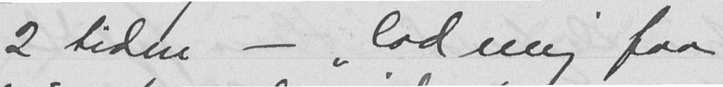2 tiden - "lad mig faa
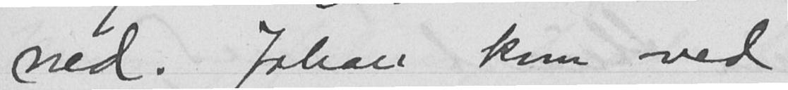ned. Johan kom ved
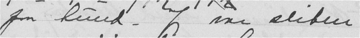paa Sund. Jeg var sliten
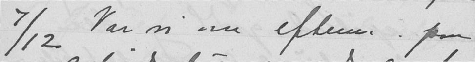7/12 Var vi om efterm. paa
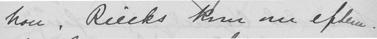han, Riecks kom om efterm.
